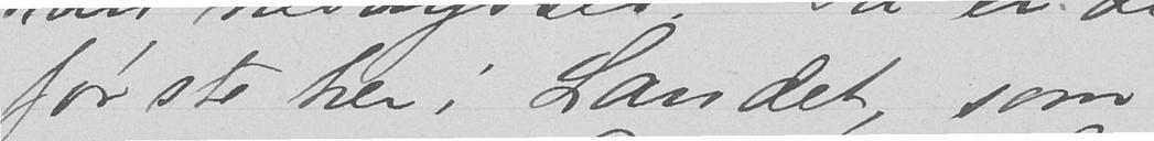første her i Landet, som
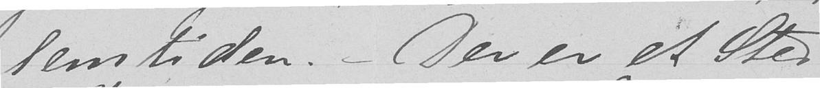lemtiden. Der er et Sted
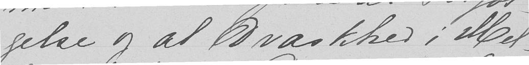gelse og al Dvaskhed i Mel-
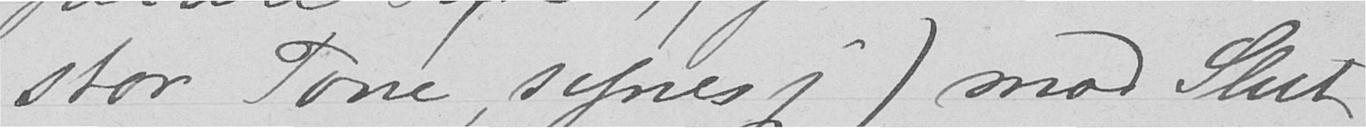stor Tone, synes jeg) mod Slut-
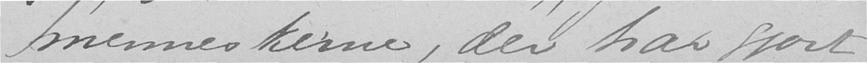menneskerne, der har gjort
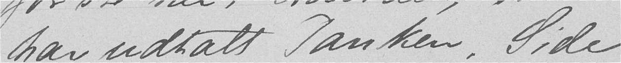har udtalt Tanken. Side
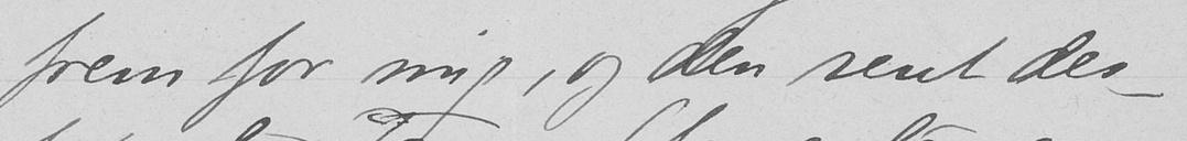frem for mig, og den rent des-
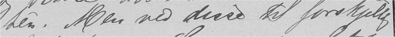ten. Men ved disse til forskjellig
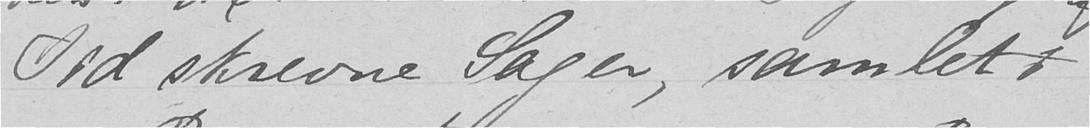Tid skrevne Sager, samlet i
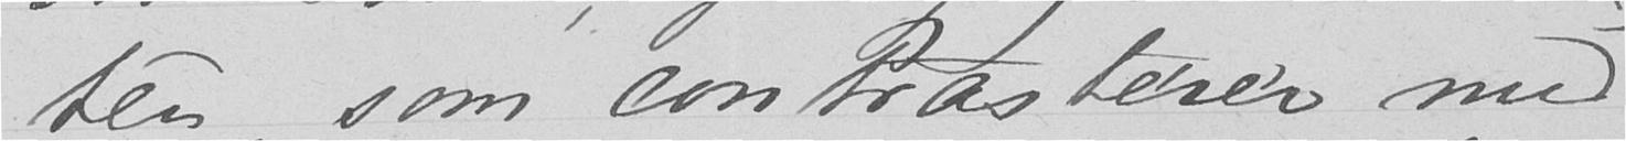ten, som contrasterer med
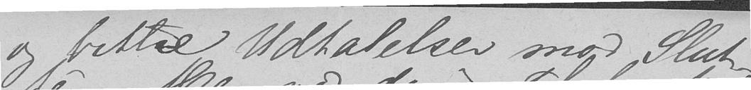og bittre Udtalelser mod Slut-
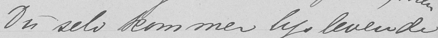Du selv kommer lys levende
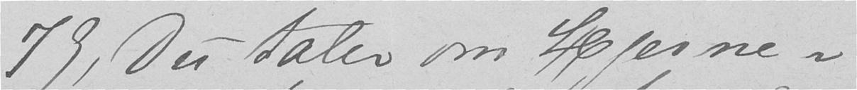79, Du taler om Hjerne-
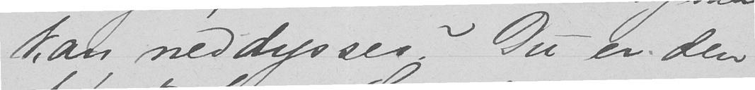kan neddysses? Du er den
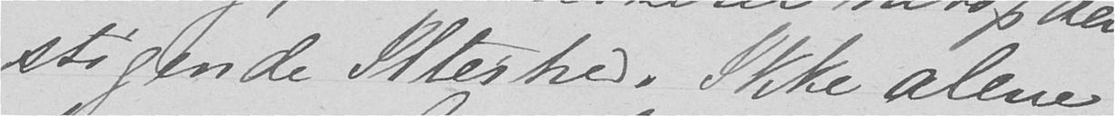stigende Ilterhed. Ikke alene
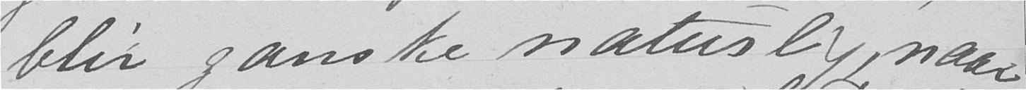blir ganske naturlig, naar
